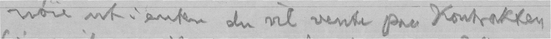nøie ut: enten du vil vente paa Kontrakten
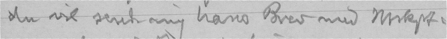du vil sende mig hans Brev med Mskpt.
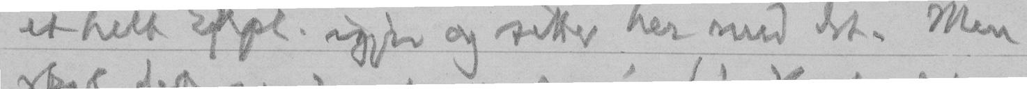et helt Ekpl. igjen og sitter her med det. Men
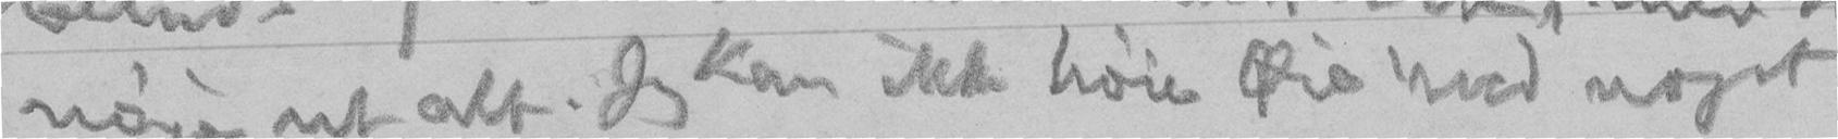nøie ut alt. Jeg kan ikke høie Øie med noget
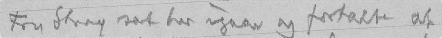Fru Stray sat her igaar og fortalte at
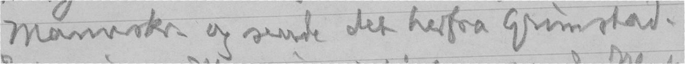Manuskr. og sende det herfra Grimstad.
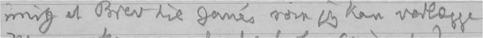mig et Brev til Janés som jeg kan vedlægge
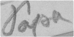Papa
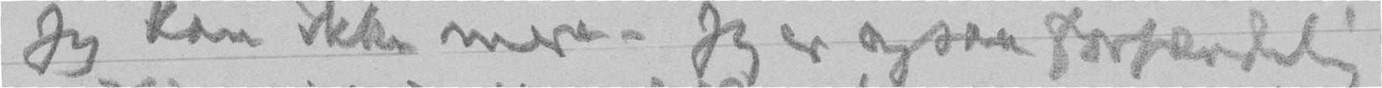Jeg kan ikke mere! Jeg er ogsaa forfærdelig
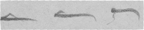- - -
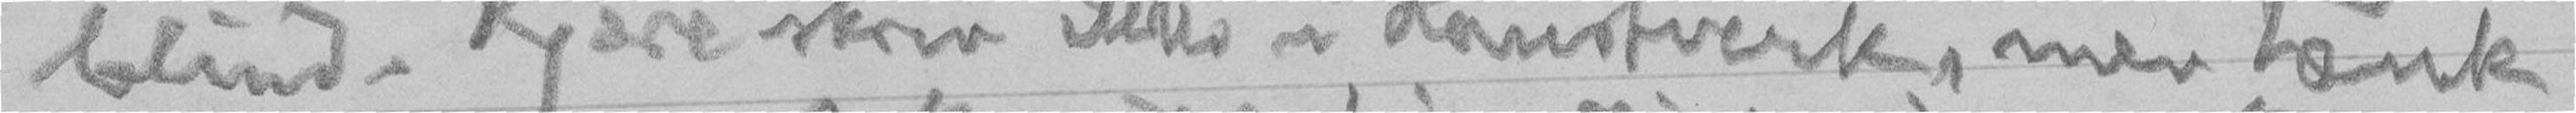blind. Kjære skriv ikke i Hanstverk, men tænk
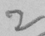2
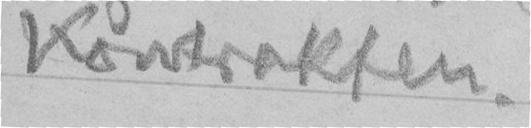Kontrakten.
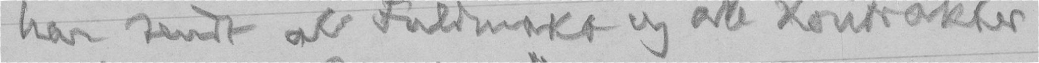har sendt al Fuldmakt og alle Kontrakter
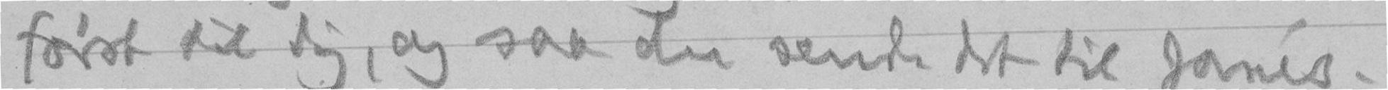først til dig, og saa du sende det til Janés.
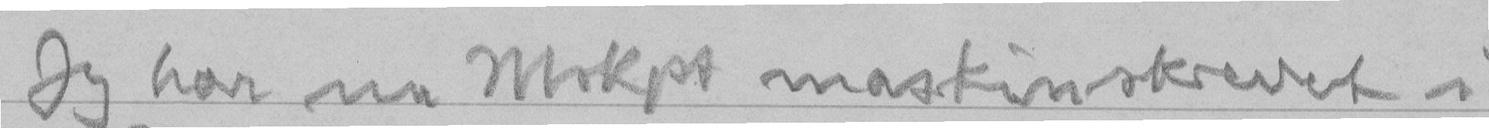Jeg har nu Mskpt maskinskrevet i
